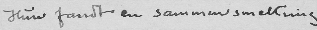Hun fandt en sammensmeltning
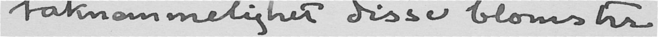taknæmmelighet disse blomster
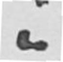i
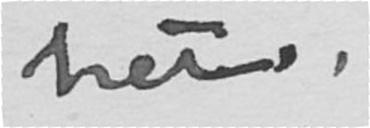het.
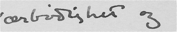ærbødighet og
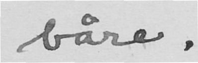båre.
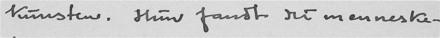kunsten. Hun fandt det menneske-
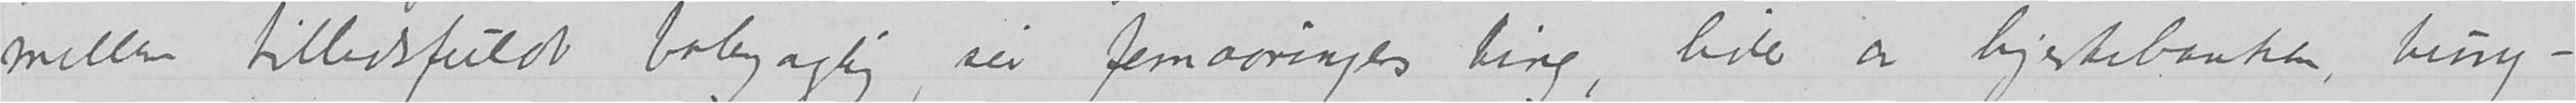mellem tillidsfuldt babyagtig, sier femaaringers ting, lider av hjertebanken, tung-
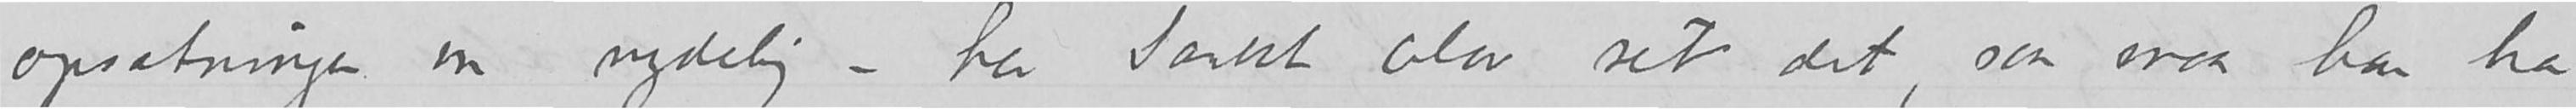opsætningen var nydelig – har Sankt Olav set det, saa maa han ha
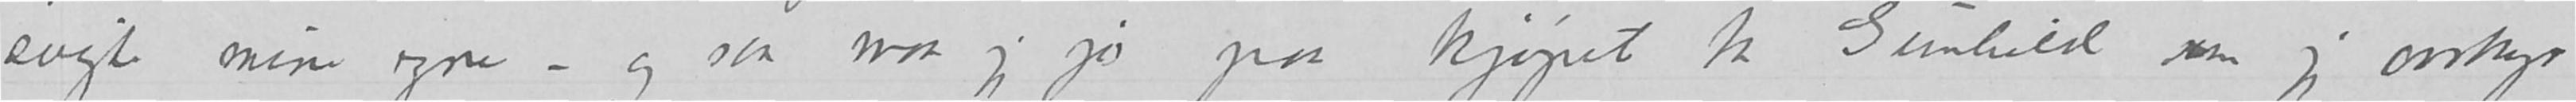svigte mine egne – og saa maa jeg jo paa kjøpet ta Gunhild som jeg avskyr
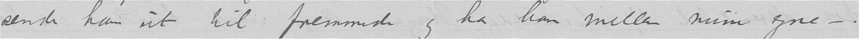sende ham ut til fremmede og ha ham mellem mine egne –.
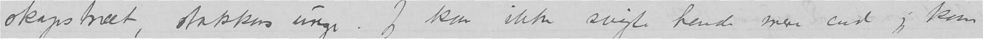skapstræet, stakkars unge.  Jeg kan ikke svigte hende mere end jeg kan
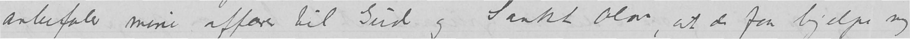anbefaler mine affærer til Gud og Sankt Olav, at de faar hjelpe mig,
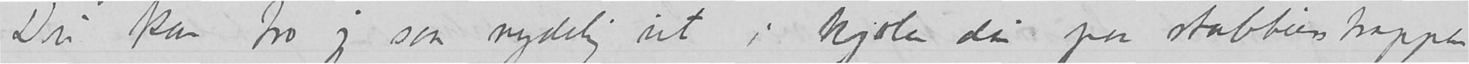Du kan tro jeg saa nydelig ut i kjolen din paa stabburstrappen
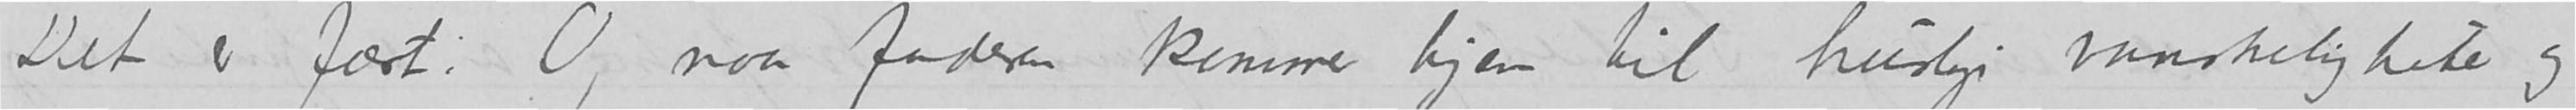Det er fært.  Og naar faderen kommer hjem til huslige vanskeligheter og
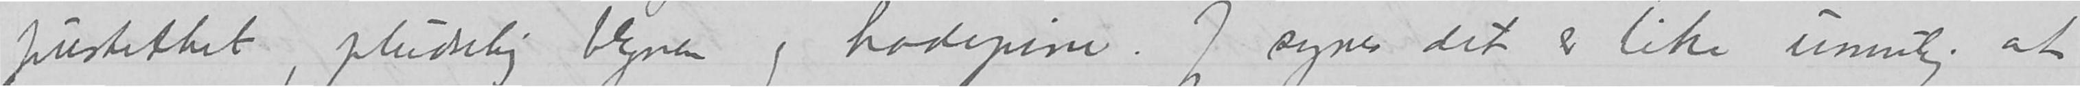pustethet, pludselig blegnen og hodepine.  Jeg synes det er like umulig at
